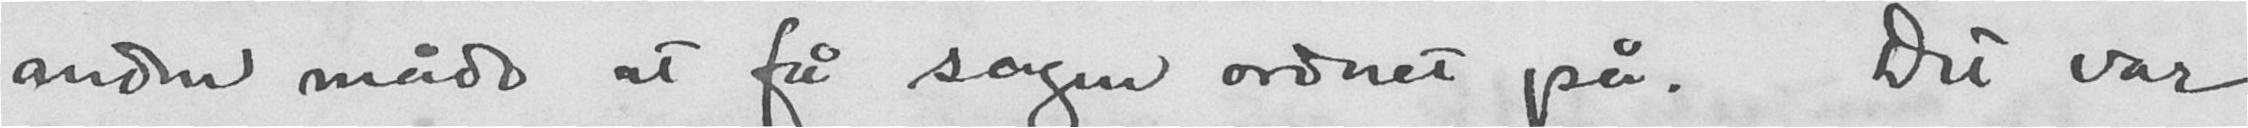anden måde at få sagen ordnet på. Det var
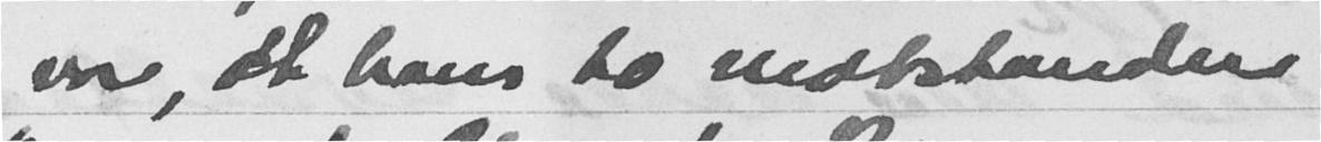var, at hans to motstandere
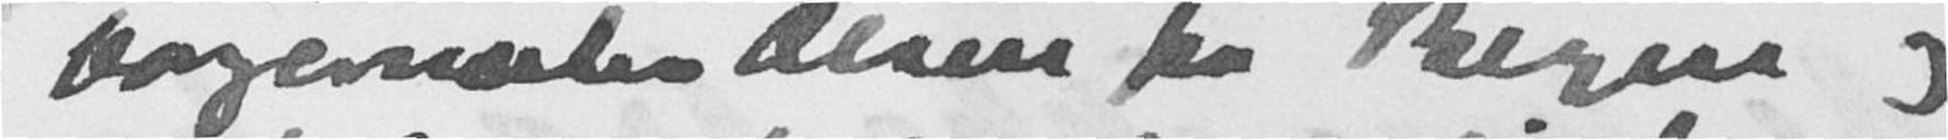borgermester Olsen fra Bergen og
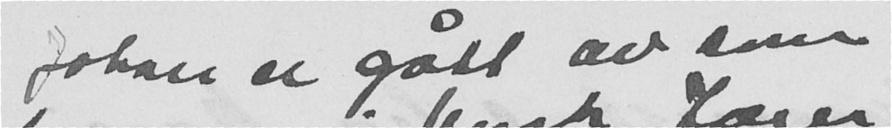Johan er gått av som
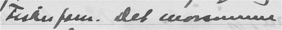Fiskeriforn. Det morsomme
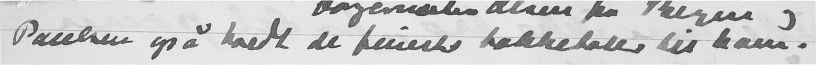Paulsen også holdt de fineste takketaler til ham.
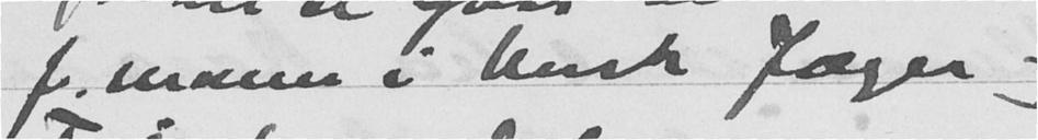f. mann i Norsk Jæger og
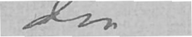din
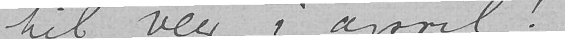til veir i april!
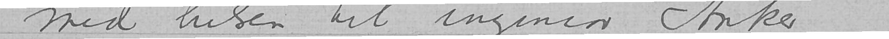med {sic} hilsen til ingeniør Anker
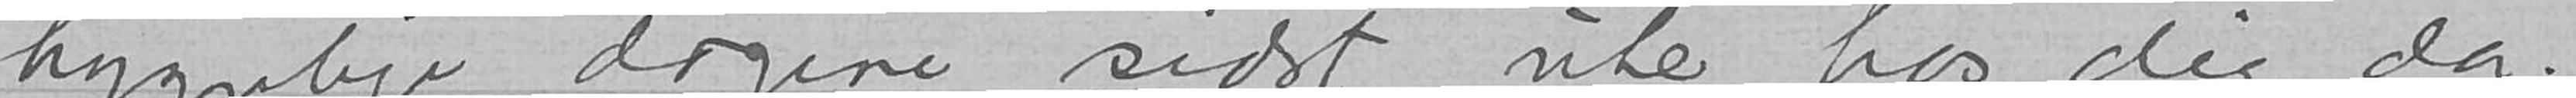Ja det var altsaa tak for de hyggelige dagene sidst ute hos dig da.
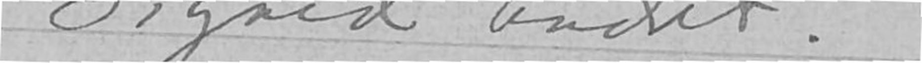Sigrid Undset
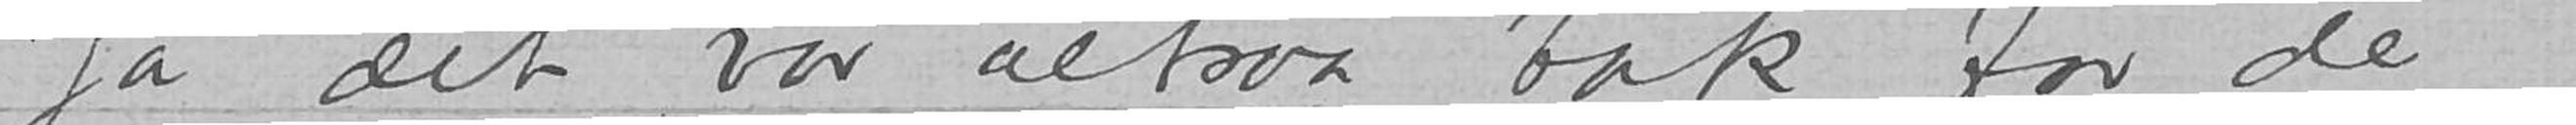Ja det var altsaa tak for de
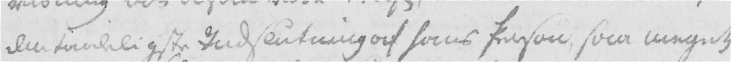den taaleligste Indslutning af hans Person, saa meget
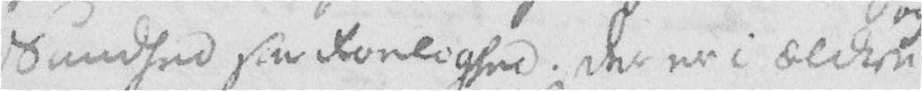Sundhed sin Roelighed. Der er i ældre
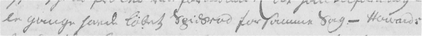le Gange havde løbet Spidsrod for samme Sag. - Howards
